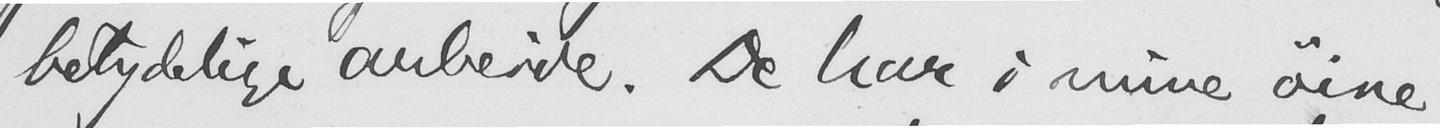betydelige arbeide. De har i mine øine
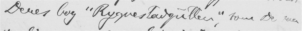Deres bog "Rygnestadgutten", som De saa
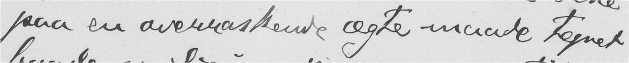paa en overraskende ægte maade tegnet
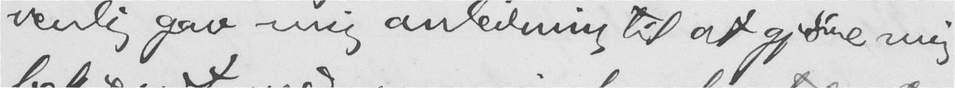venlig gav mig anledning til at gjøre mig
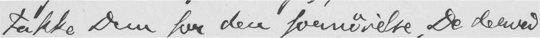takke Dem for den fornøielse, De derved
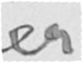er
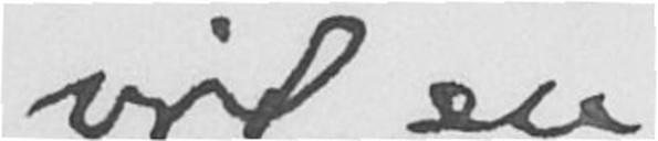vil en
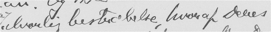alvorlig bestræbelse, hvoraf Deres
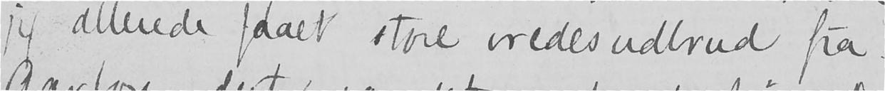jeg allerede faaet store vredesudbrud fra
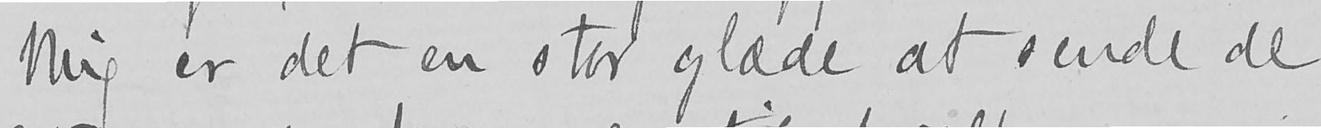Mig er det en stor glæde at sende de
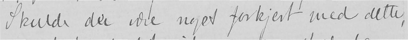Skulde der være noget forkjert med dette,
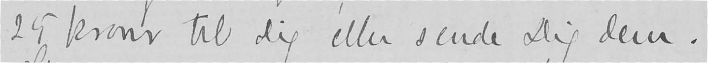25 kroner til dig eller sende Dig dem.
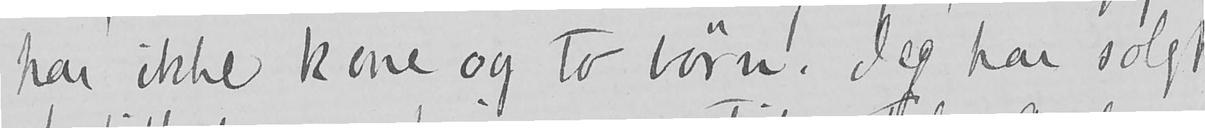har ikke kone og to børn. Jeg har solgt
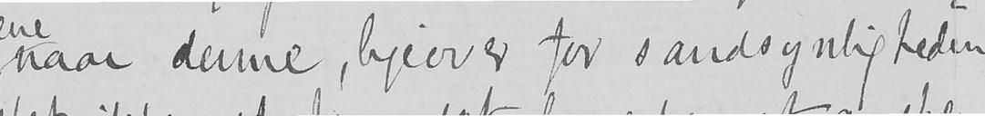naar denne, ligeover for sandsynligheden
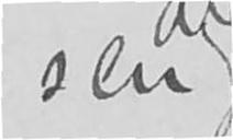sen
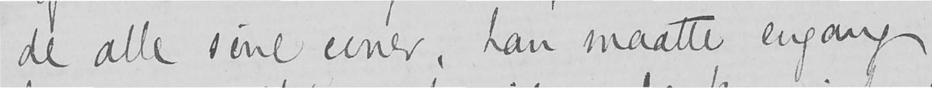de alle sine evner, han maatte engang
